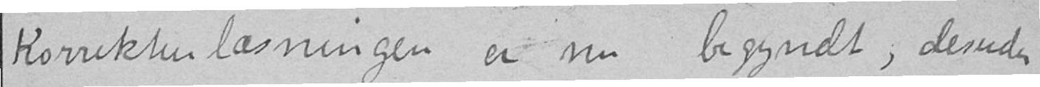Korrekturlæsningen er nu begyndt, desuden
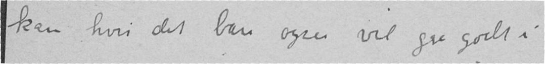kan hvis det bare ogsaa vil gaa godt i
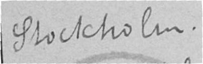Stockholm.
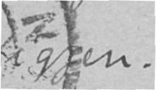igjen.
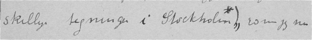skillige tegninger i Stockholm*), som jeg nu
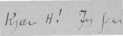Kjære H! Jeg faar
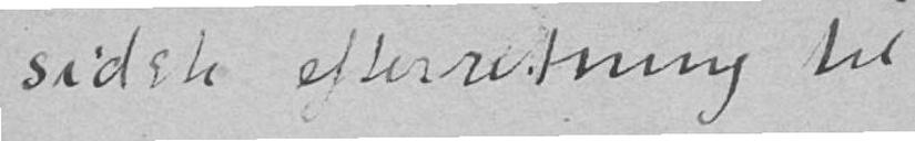sidste efterretning til
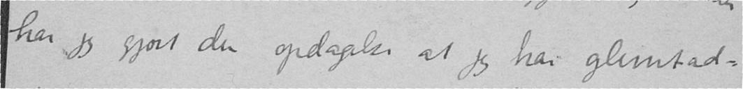har jeg gjort den opdagelse at jeg har glemt ad-
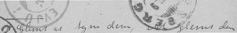*Glemt at tegne dem, ikke glemt dem
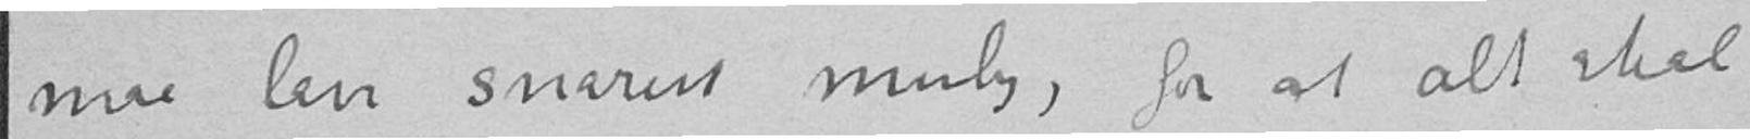maa lave snarest mulig, for at alt skal
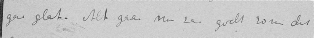gaa glat. Alt gaar nu saa godt som det
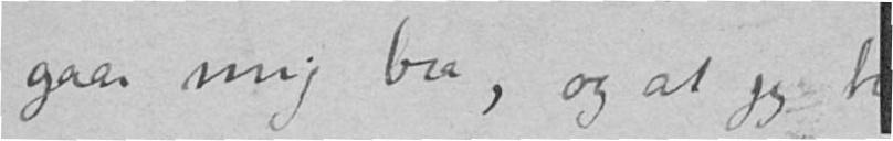gaar mig bra, og at jeg jo
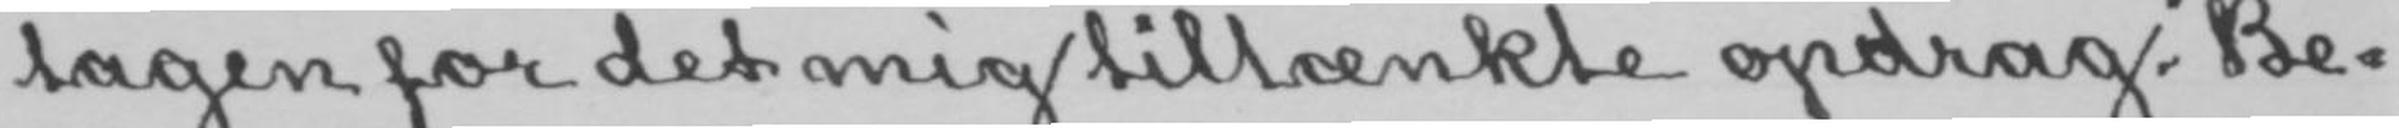tagen for det mig tiltænkte opdrag. Be-
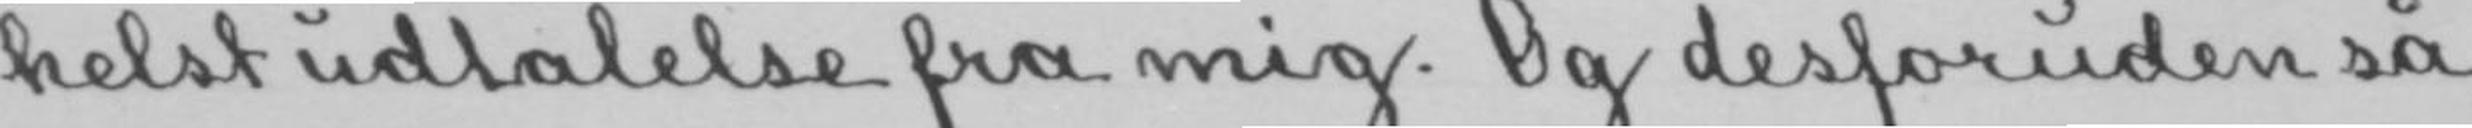helst udtalelse fra mig. Og desforuden så
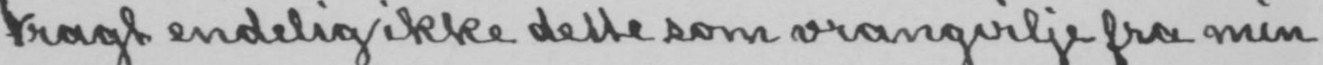tragt endelig ikke dette som vrangvilje fra min
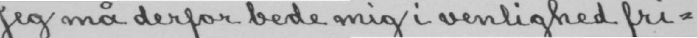Jeg må derfor bede mig i venlighet fri-
